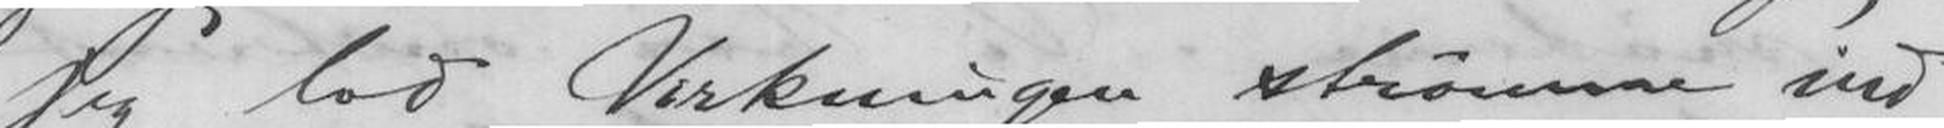jeg lod Virkningen strømme ind
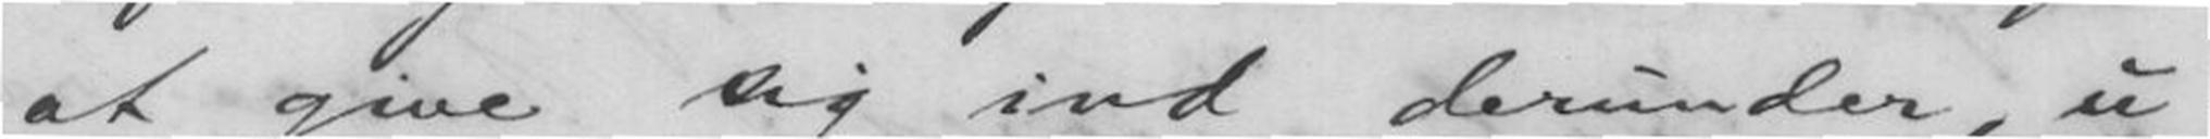at give sig ind derunder, u-
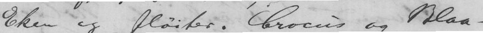Eken og fløiter. Crokus og Blaa-
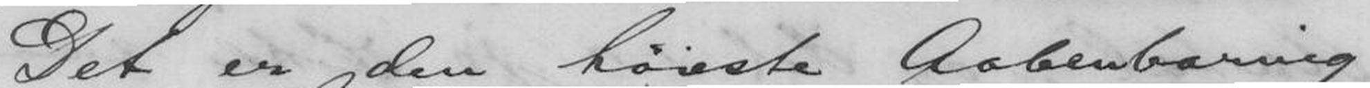Det er den høieste Aabenbaring
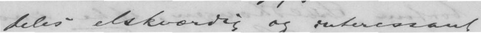deles elskværdig og interessant
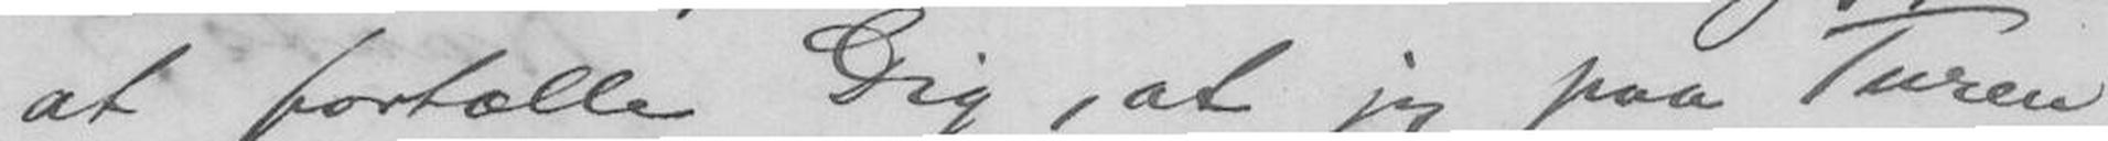at fortælle Dig, at jeg paa Turen
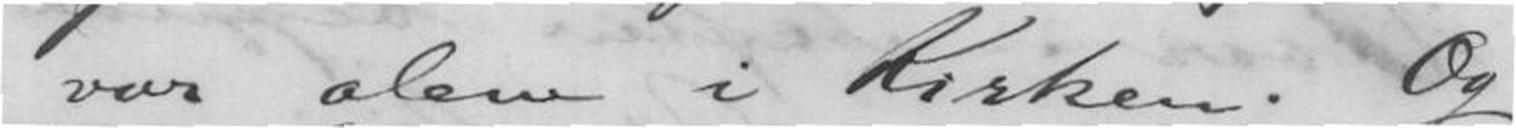var alene i Kirken. Og
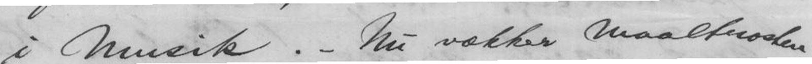i Musik.- Nu vækker Maaltrosten
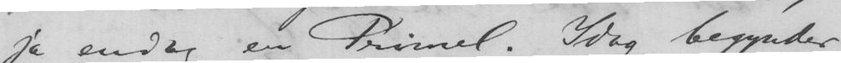ja endog en Primel. Idag begynder
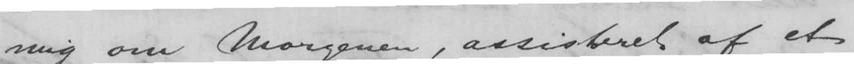mig om Morgenen, assisteret af et
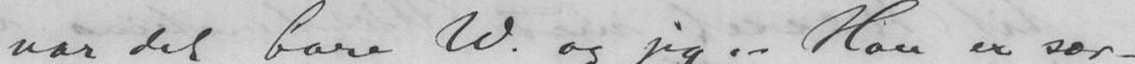var det bare W. og jeg. - Han er sær-
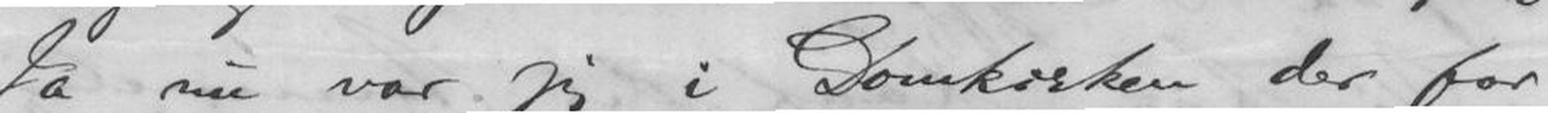Ja nu var jeg i Domkirken der for
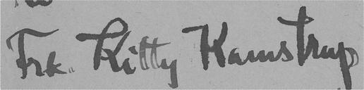Frk Kitty Kamstrup
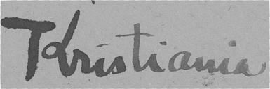Kristiania
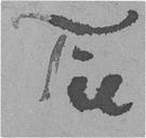Til
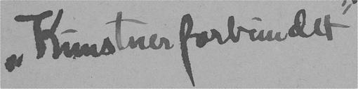"Kunstnerforbundet"
